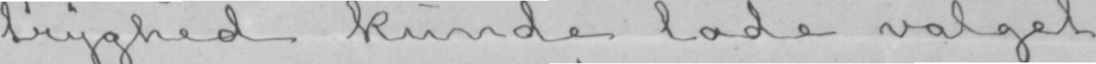tryghed kunde lade valget
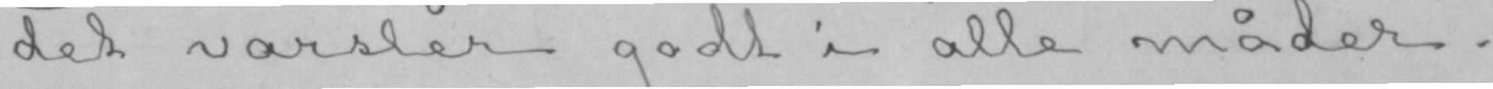det varsler godt i alle måder.
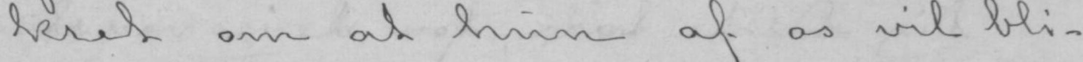kret om at hun af os vil bli-
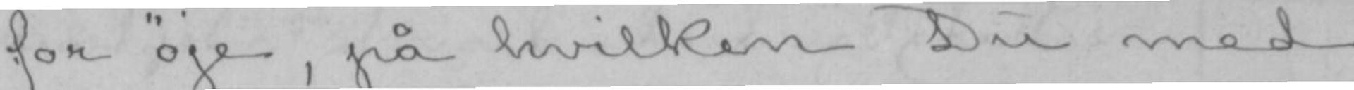for øje, på hvilken Du med
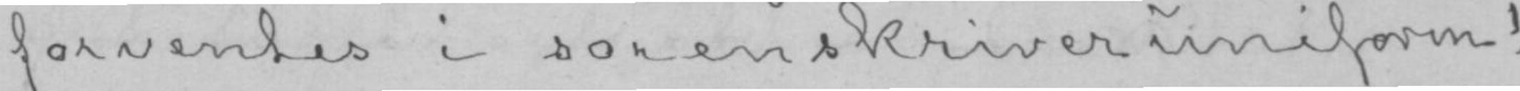forventes i sorenskriveruniform!
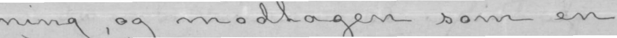ning, og modtagen som en
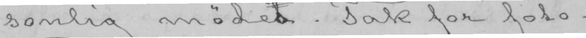sonlig mødets. Tak for foto-
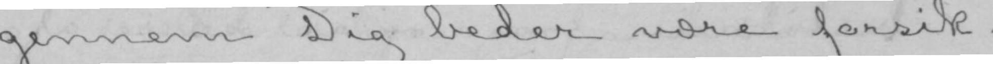gennem Dig beder være forsik-
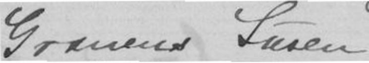Granens Susen
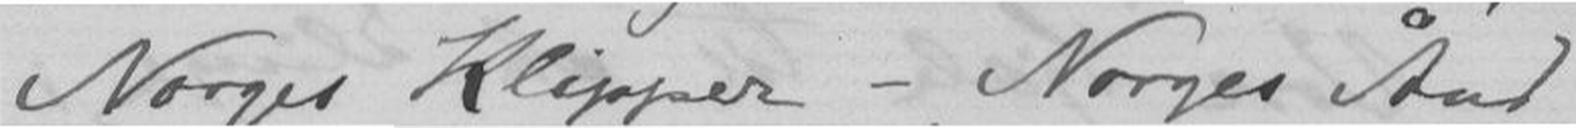Norges Klipper - Norges Ånd
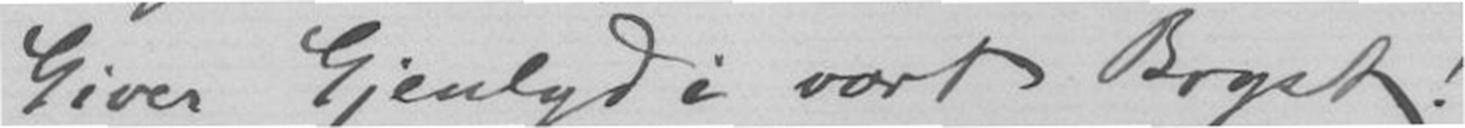Giver Gjenlyd i vort Bryst!
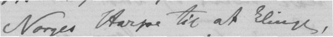Norges Harpe til at klinge,
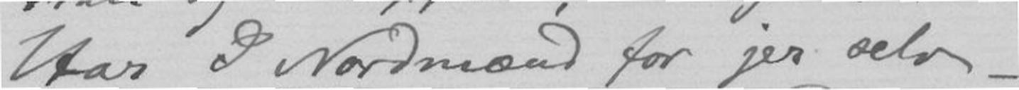Har I Nordmænd for jer selv -
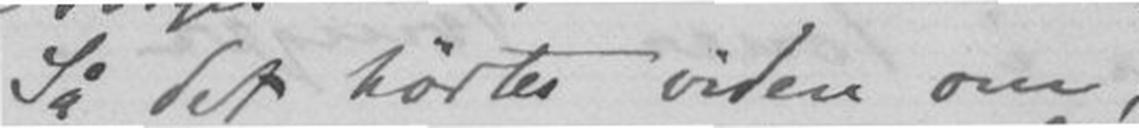Så det hørtes viden om,
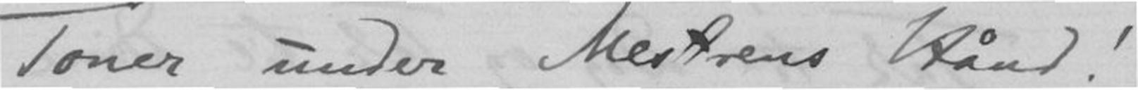Toner under Mestrens Hånd!
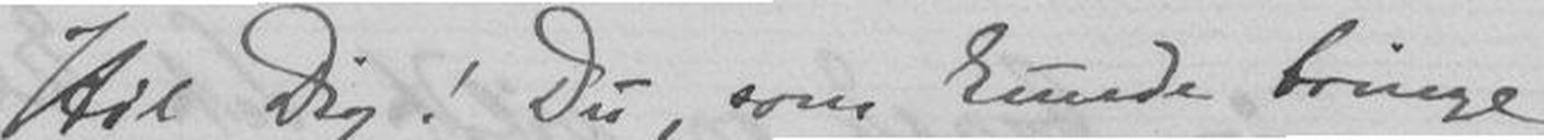Hei Dig! Du, som kunde bringe
